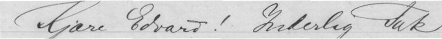Kjære Edvard! Inderlig Tak
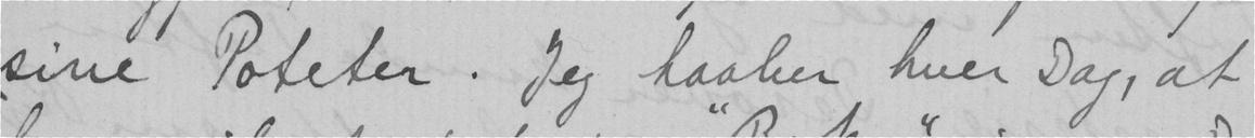sine Poteter. Jeg haaber hver Dag, at
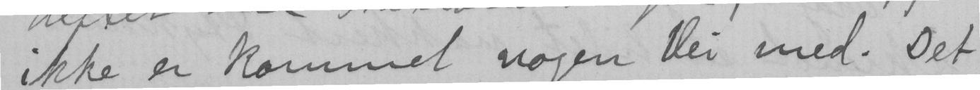ikke er kommet nogen vei med. Det
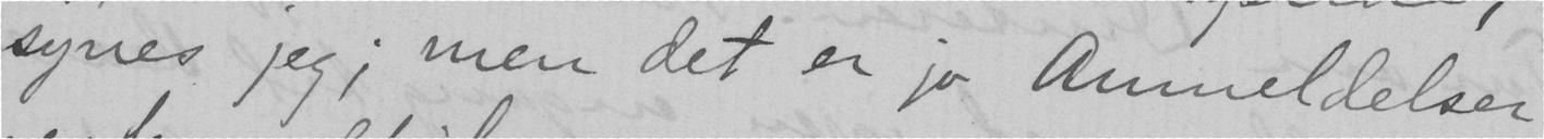synes jeg; men det er jo anmeldelser
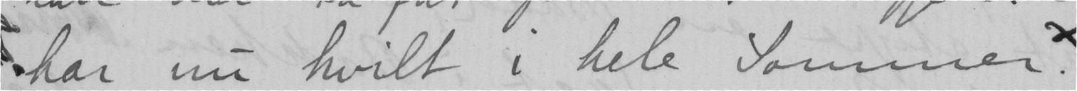har nu hvilt i hele Sommer.
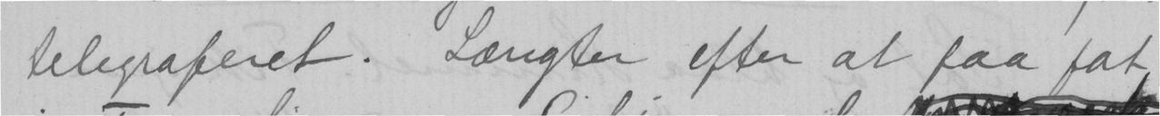telegraferet. Længter efter at faa fat
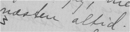næsten altid.
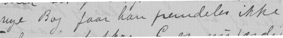nye Bog faar han fremdeles ikke
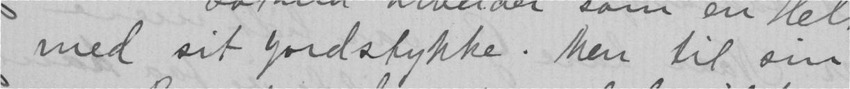med sit Jordstykke. Men til sin
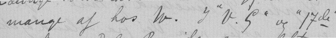mange af hos W. I "V. G." og "17de"
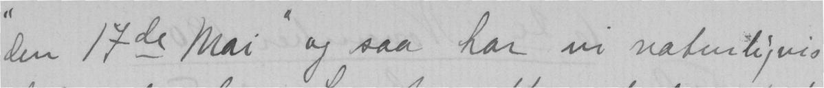"den 17de mai" og saa har vi naturligvis
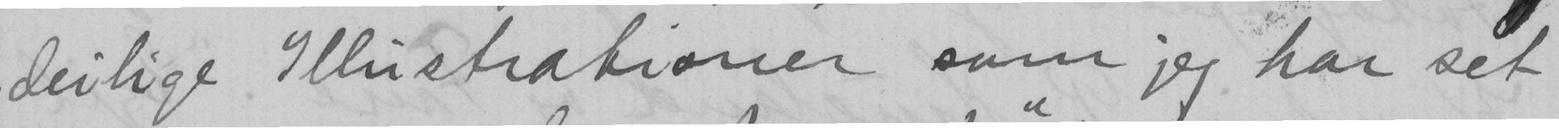deilige Illustrationer som jeg har set
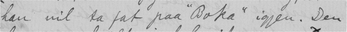han vil ta fat paa "Boka'" igjen. Den
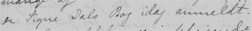er Signe Dals Bog idag anmeldt.
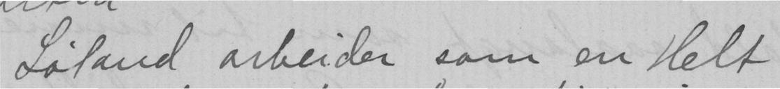Løland arbeider som en Helt
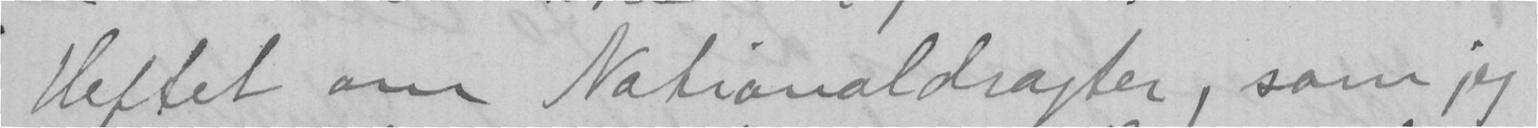Heftet om Nationaldragter, som jeg
